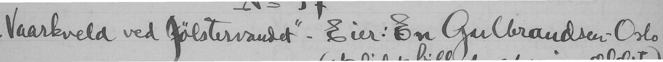"Vaarkveld ved Jølstervandet". Eier: En Gulbrandsen - Oslo
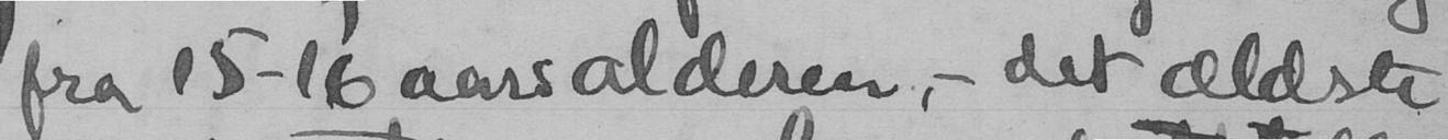fra 15-16 aars alderen, - det ældste
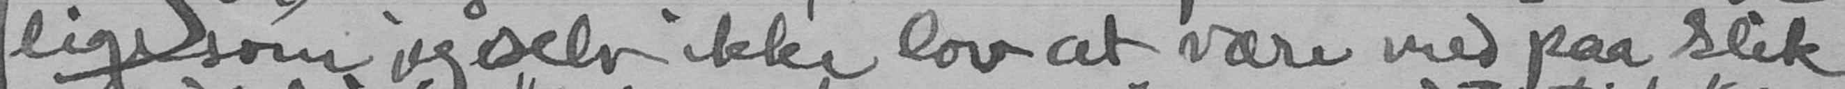ligssom jeg selv ikke lov at være med paa slik
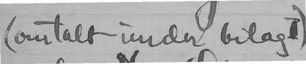(omtalt under bilag I)
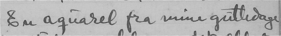En aquarel fra mine guttedage
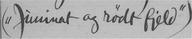("Juninat og rødt fjeld")
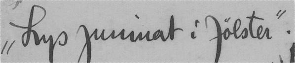"Lys juninat i Jølster"
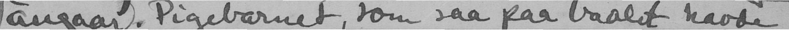angaar. Pigebarnet, som saa paa baalet havde
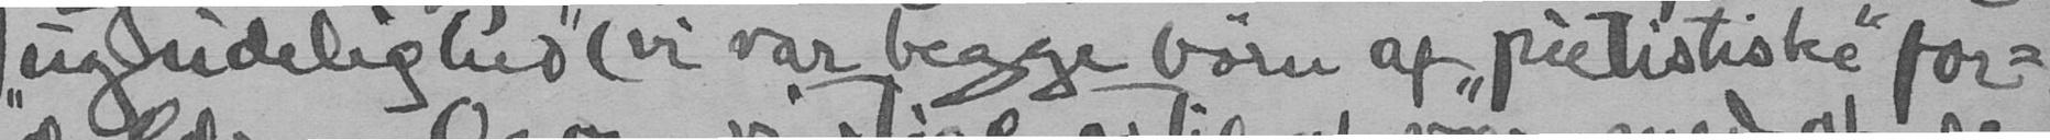ugudelighed (vi var begge børn af "pietistiske" for-
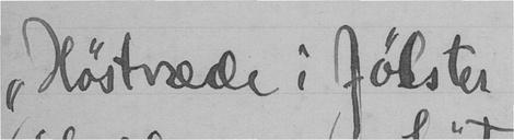"Høstvæde i Jølster
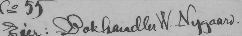Eier: Bokhandler W. Nygaard.
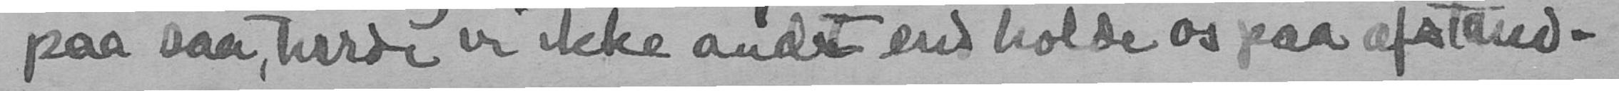paa saa, turde vi ikke andet end holde os paa afstand-
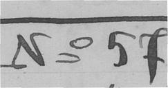No 57
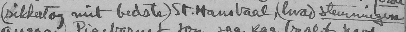(sikkert og mit bedste) St. Hansbaal, (hvad stemningen
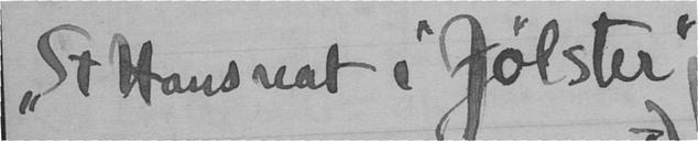"St Hansnat i Jølster"
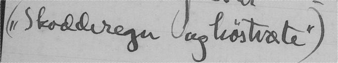("Skodderegn og høstvæte")
